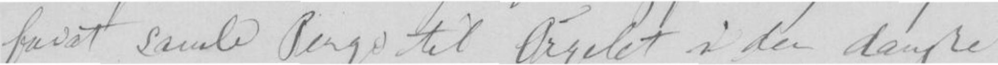først samle Penge til Orgelet i den danske
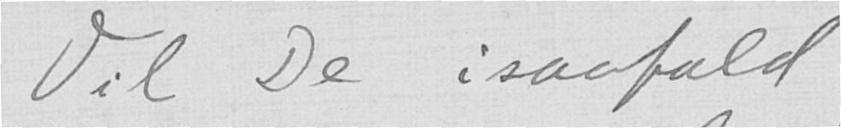Vil De isaafald
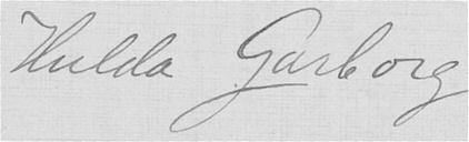Hulda Garborg
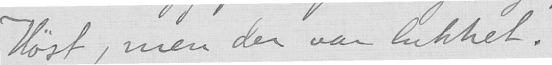Høst, men der var lukket.
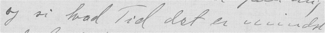og si hvad Tid det er mindst
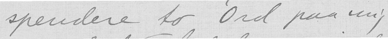spendere to Ord paa mig
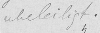ubeleiligt.
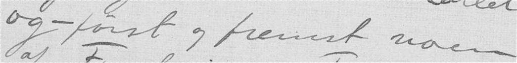og — først og fremst noen
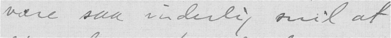være saa inderlig snil at
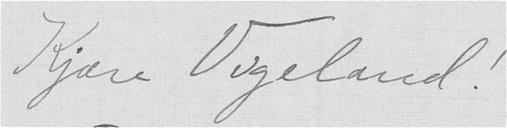Kjære Vigeland!
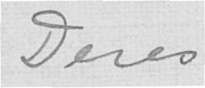Deres
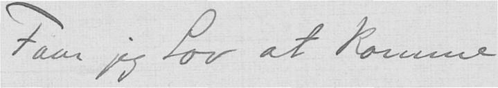Faar jeg Lov at komme
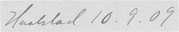Hvalstad 10.9.09
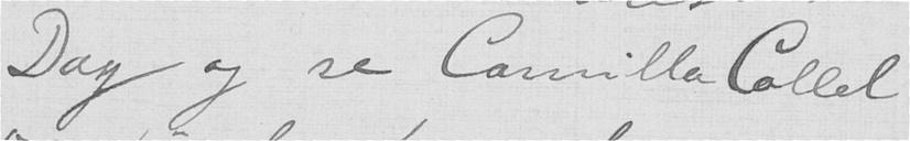Dag og se Camilla Collet
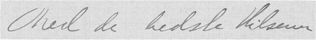Med de bedste Hilsener
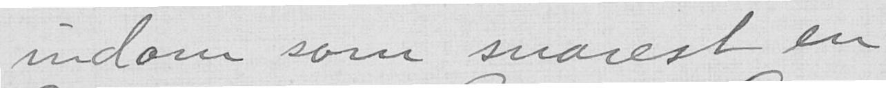indom som snarest en
